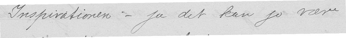Inspirationen - ja det kan jo være
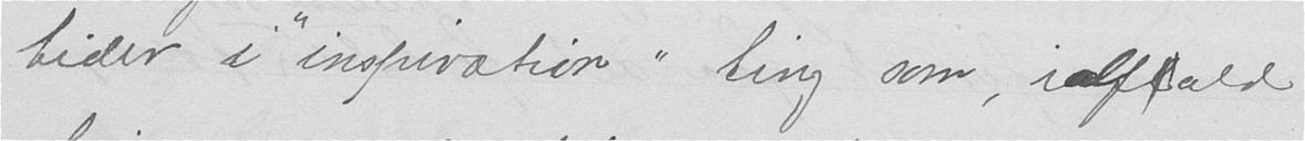tider i "inspiration" ting som, ialfald
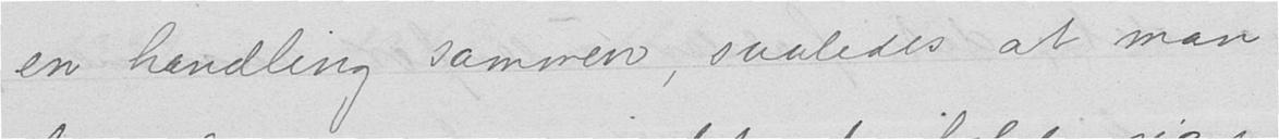en handling sammen, saaledes at man
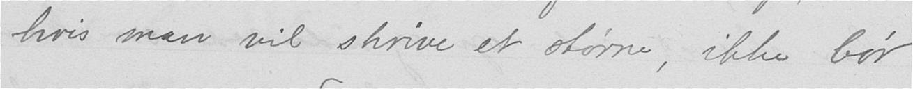hvis man vil skrive et større, ikke bør
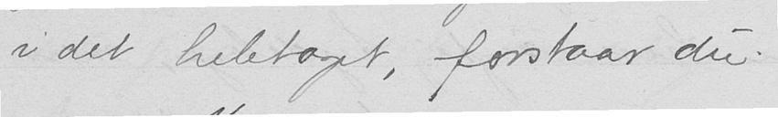i det heletaget, forstaar du.
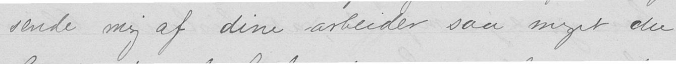sende mig af dine arbeider saa meget du
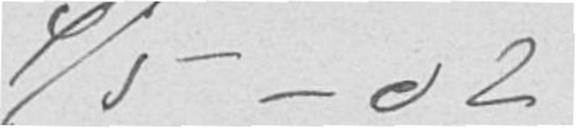4/5 - 02
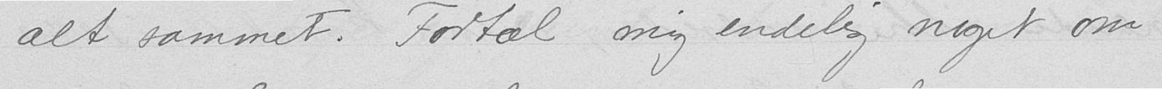alt sammet. Fortæl mig endelig noget om
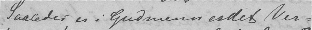Saaledes er i Gudmennesket Ver-
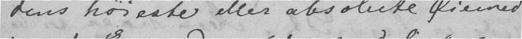dens høieste eller absolute Øiemed
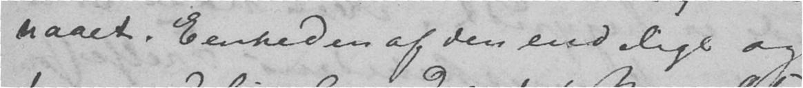naaet. Eenheden af den endelige og
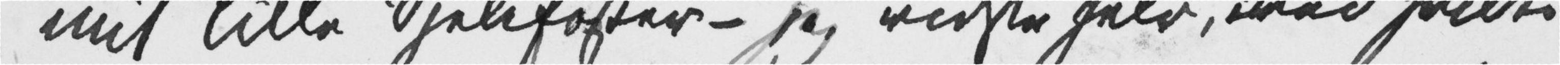mit lille Sjelefoster – jeg vidste selv, med hvilke
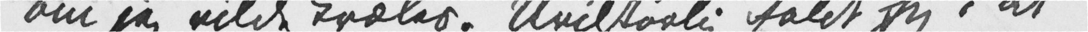om jeg vilde kvæles. Uvilkårlig faldt jeg i at
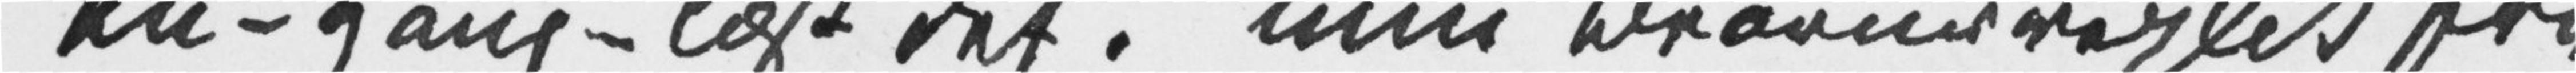en – gang – læst det!» min Bravurreplik fra
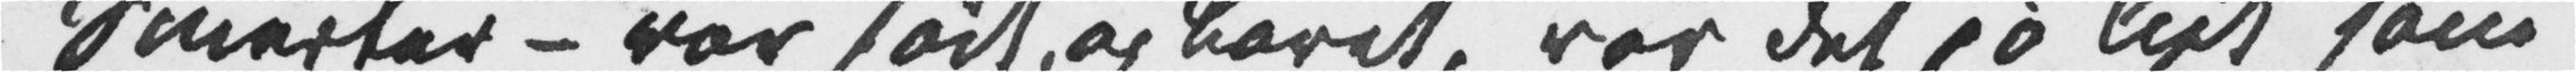Smerter – var født og båret, var det jo lidt som
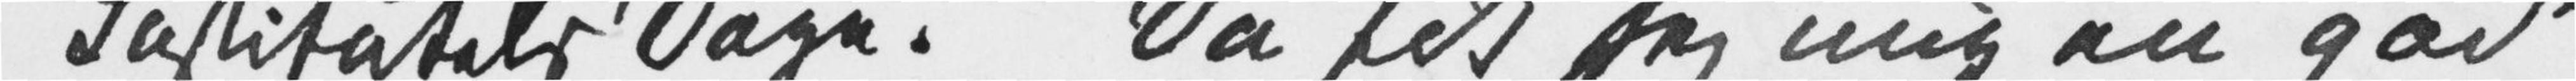Institutets Dage. Så fik jeg mig en god
